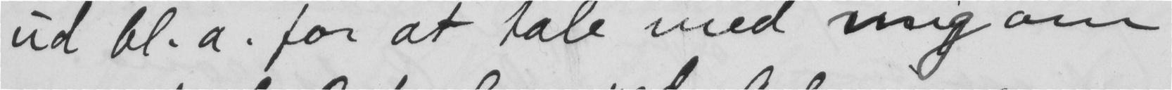ud bl.a. for at tale med mig om
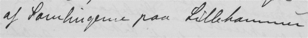af Samlingerne paa Lillehammer
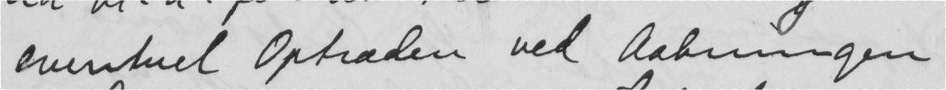eventuel Optræden ved Aabningen
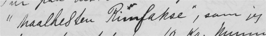"Maalhesten Rimfakse", som jeg
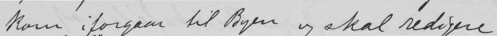kom iforgaar til Byen og skal redigere
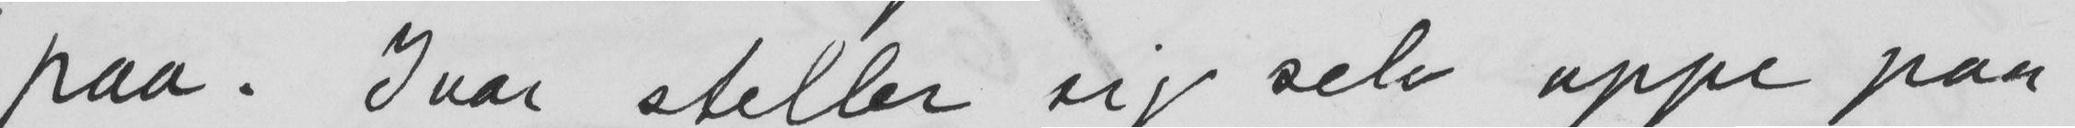paa. Ivar steller sig selv oppe paa
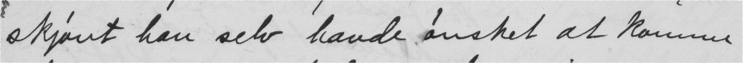skjont han selv havde ønsket at komme
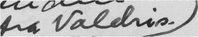fra Valdris.
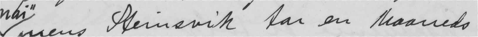mens Steinsvik tar en Maaneds
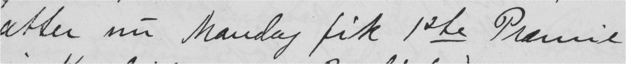atter nu Mandag fik 1ste Præmie
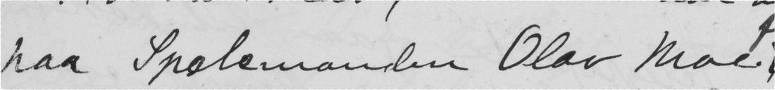paa Spelemanden Olav Moe
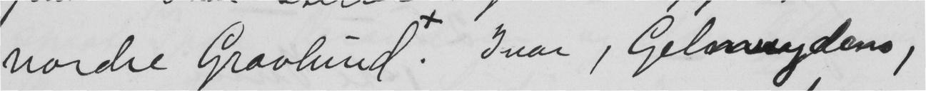nordre Gravlund*. Ivar, Gelogdens,
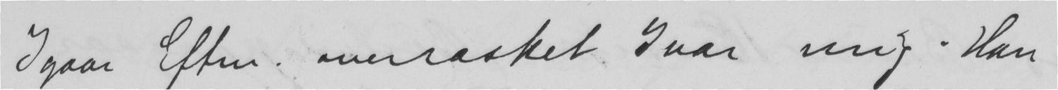Igaar Eften. overrasket Ivar mig. Han
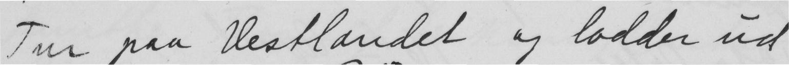Tur paa Vestlandet og lodder ud
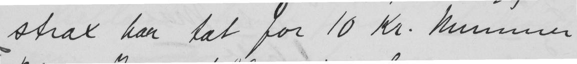strax har tat for 10 Kr. Nummer
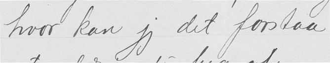hvor kan jeg det forstaa,
